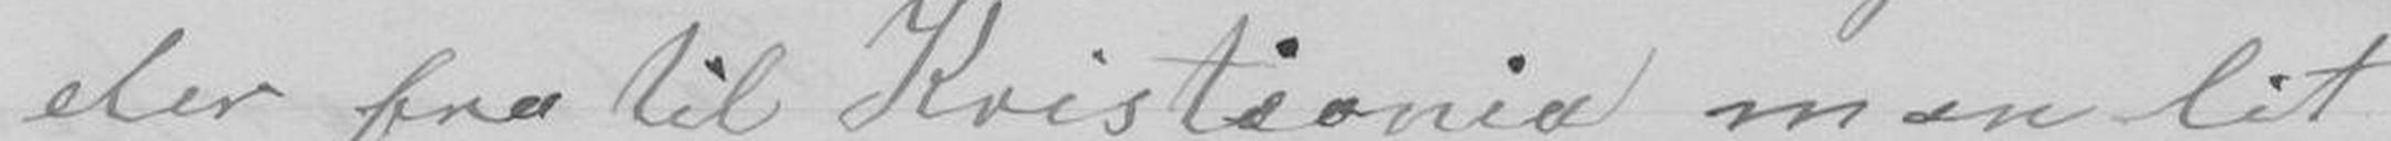der fra til Kristiania men lit
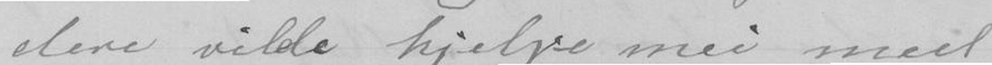dere vilde hjelpe mei med
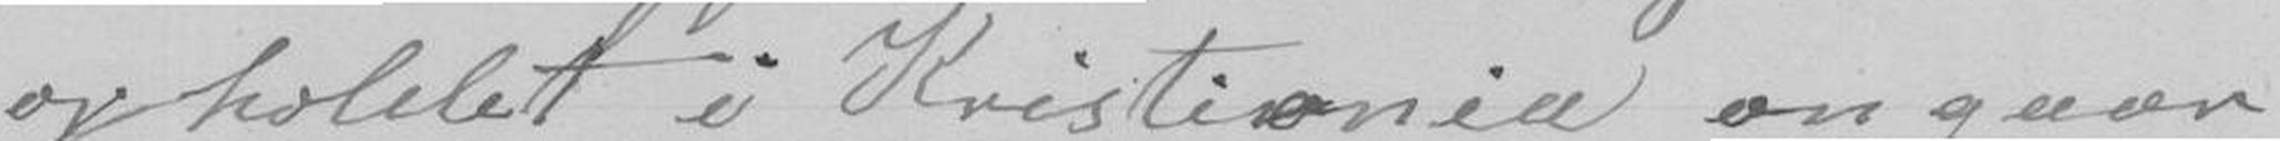opholdet i Kristiania angaar
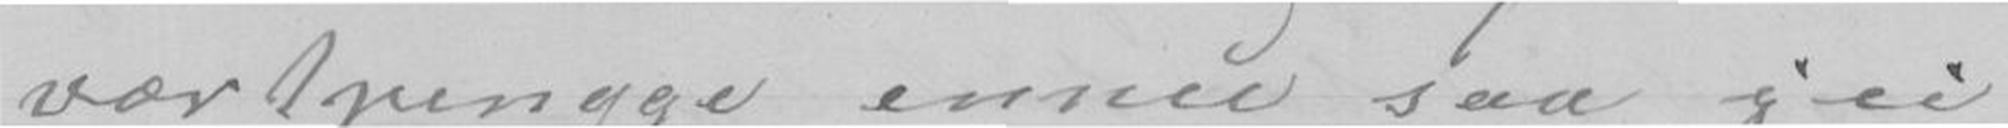værtpengge ennu saa jei
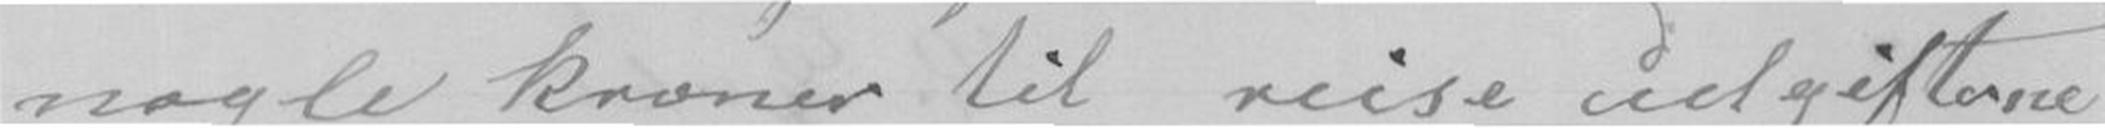nogle kroner til reise udgifterne
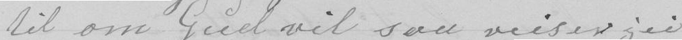til om Gud vil saa reiser jei
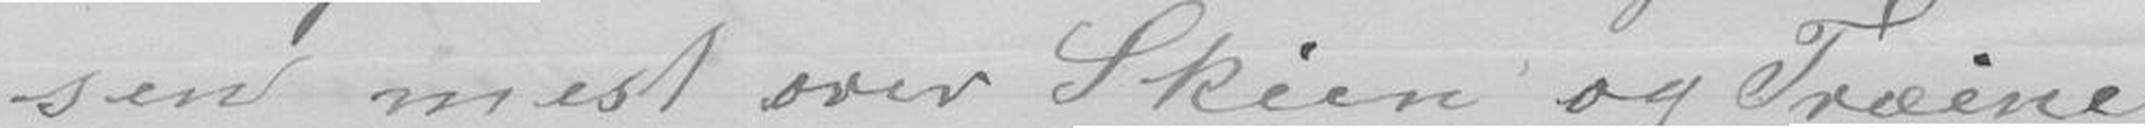sen mest over Skien og Træine
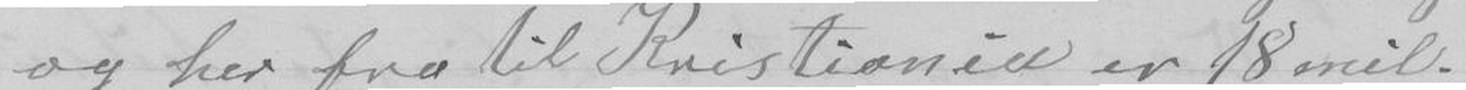og her fra til Kristiania er 18 mil.
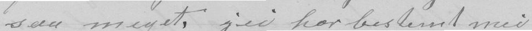saa meget. jei har bestemt mei
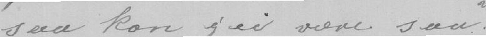saa kan jei være saa
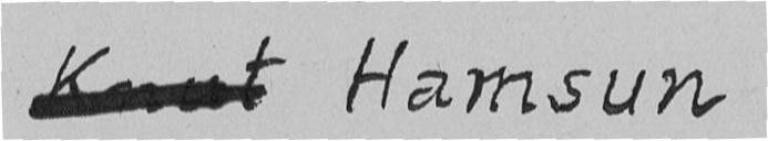Knut Hamsun
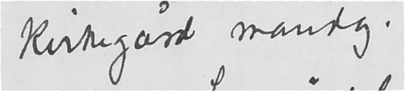kirkegard mandag.
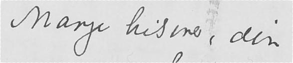Mange hilsener, din
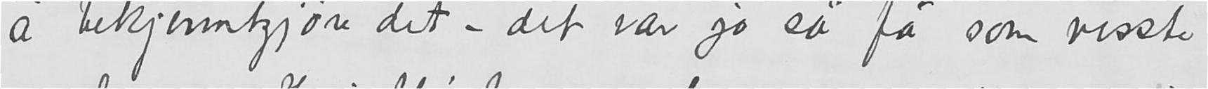å bekjentgjøre det – det var jo så få som visste
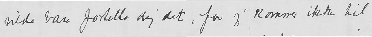vilde bare fortelle dig det, for jeg kommer ikke til
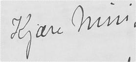Kjære Nini,
